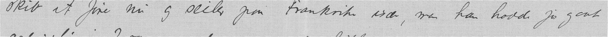skib at føre nu og seiler paa Frankrike især, men han hadde jo gaat
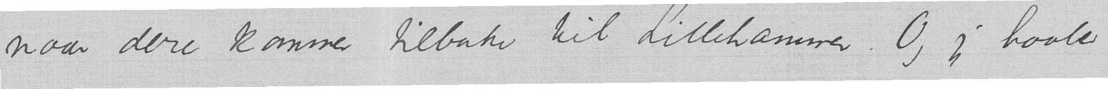naar dere kommer tilbake til Lillehammer. Og jeg haaber
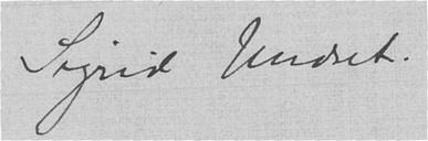Sigrid Undset.
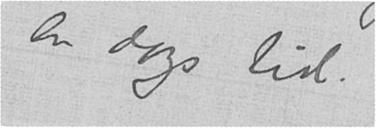en dags tid.
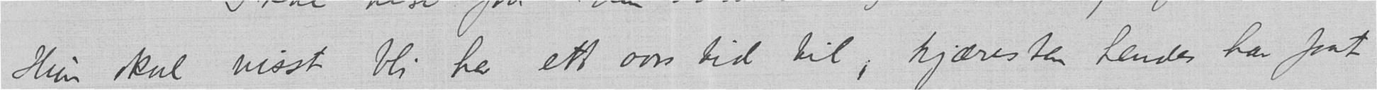Hun skal visst bli her ett aars tid til; kjæresten hendes har faat
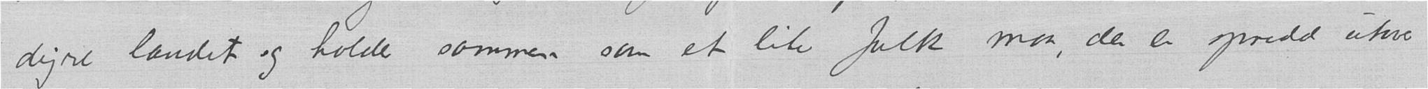digre landet og holde sammen som et lite folk maa, der er spredd utover
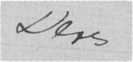Deres
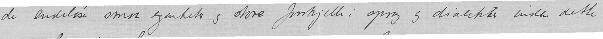de endeløse smaa egenheter og store forskjelle i sprog og dialekter inden dette
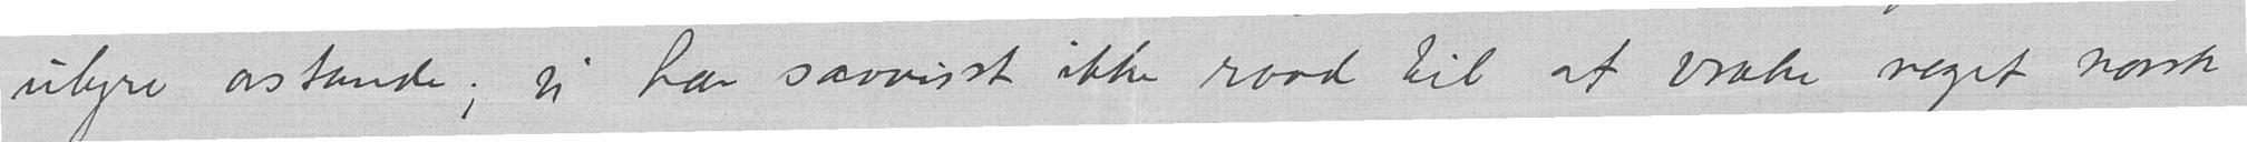uhyre avstande; vi har saavisst ikke raad til at vrake noget norsk
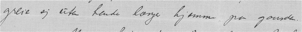greie sig uten hende længer hjemme paa gaarden.
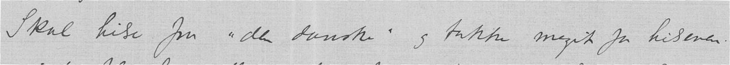Skal hilse fra "den danske" og takke meget for hilsenen.
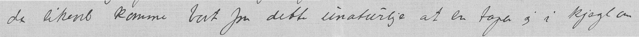da likevel komme bort fra dette unaturlige at en taper sig i kjegl om
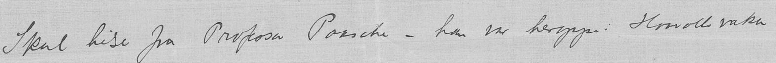Skal hilse fra Professor Paasche – han var heroppe i Haraldsvaken
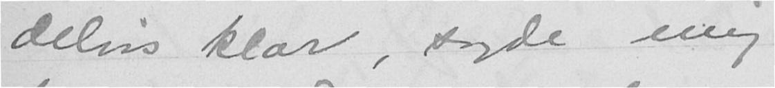delvis klar, sagde mig
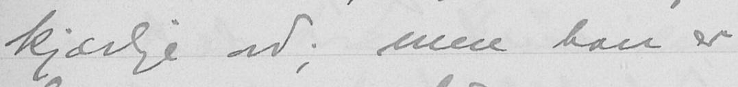kjærlige ord; men han er
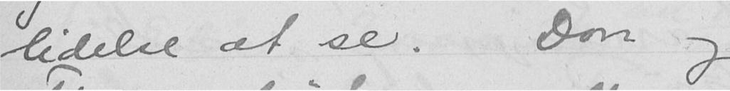lidelse at se. Don og
